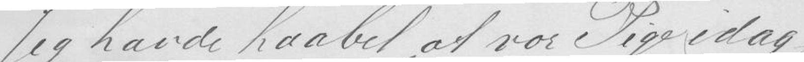Jeg havde haabet at vor Pige idag
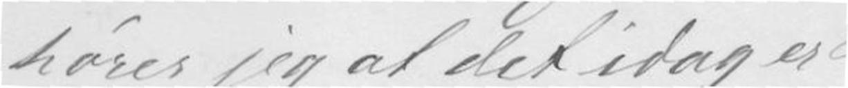hører jeg at det idag er
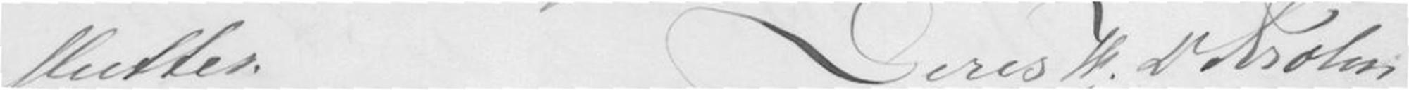Deres W.D. Krohn
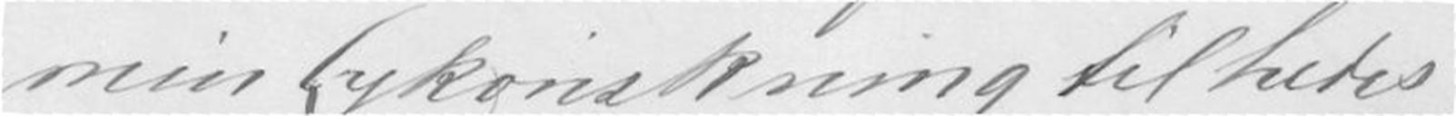min Lykønskning tilbedes
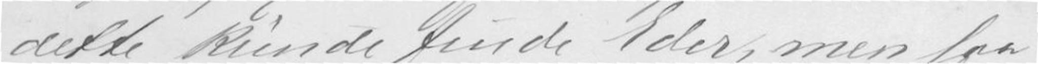dette kunde finde Eder, men saa
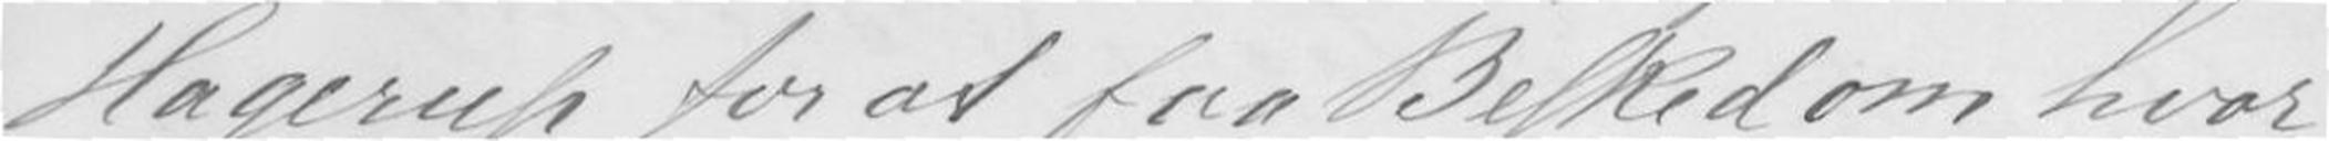Hagerup for at faa Besked om hvor
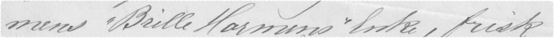men "Brille Hormens" Enke frisk
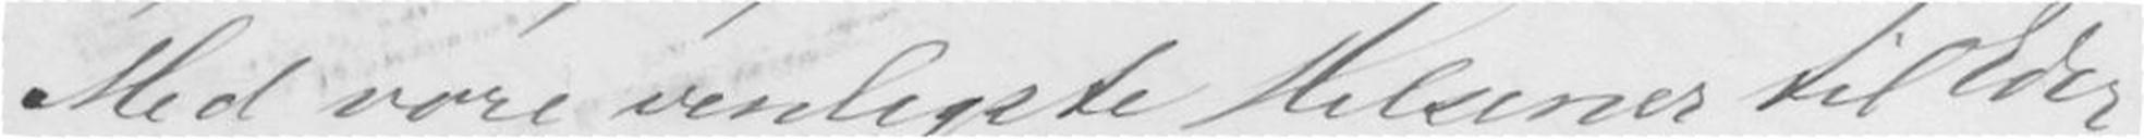Med vore venligste Hilsener til Eder
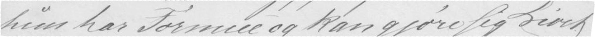hun har formue og kan gjøre sig Livet
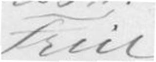Frue
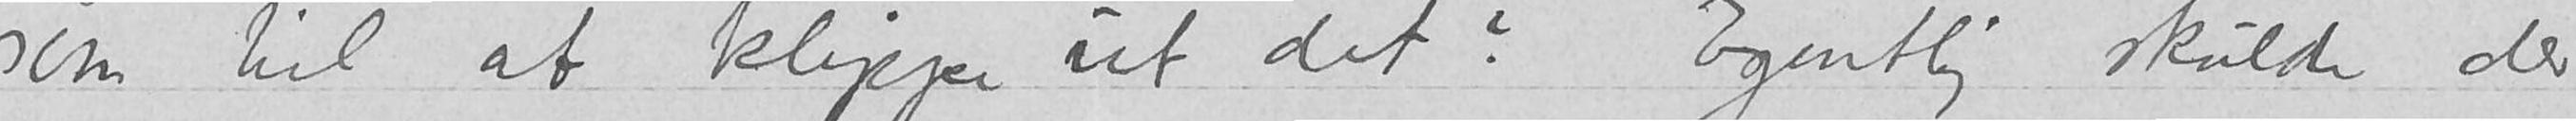som til at klippe ut det? Egentlig skulde der
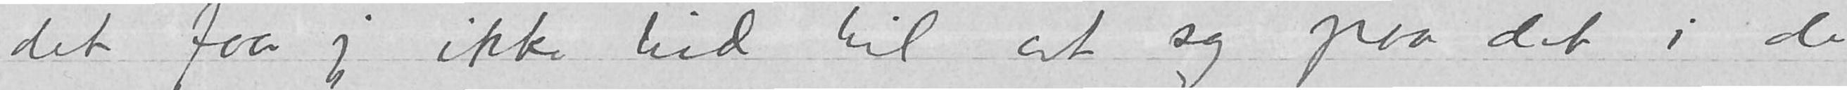det faar jeg ikke tid til at sy paa det i de
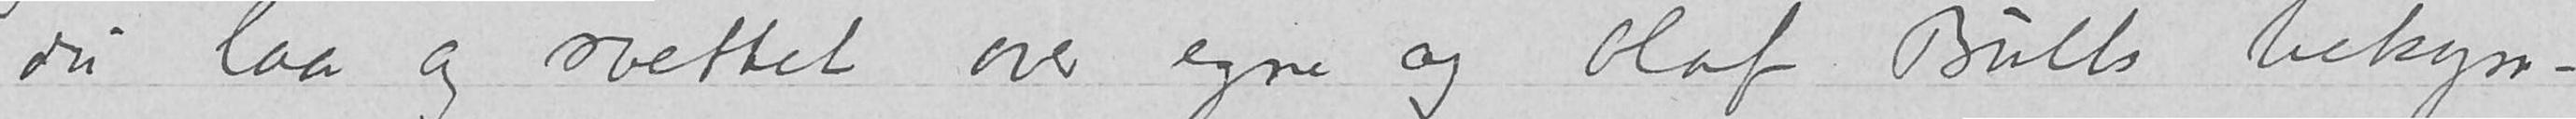du laa og svettet over egne og Olaf Bulls bekym-
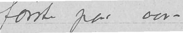første par aar –
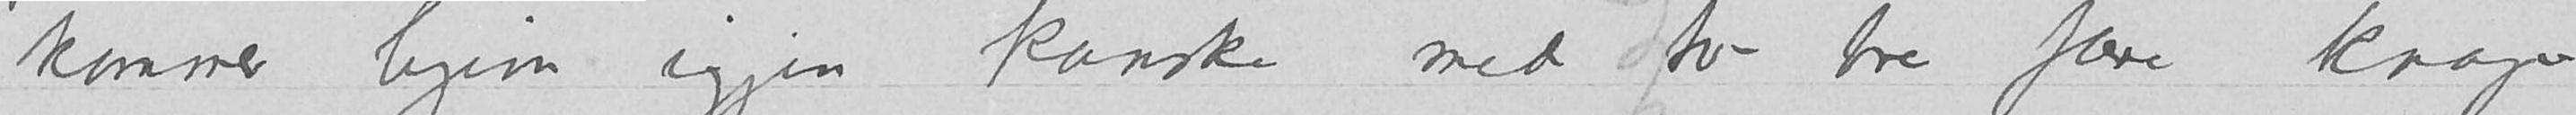kommer hjem igjen kanske med to-tre flere knap
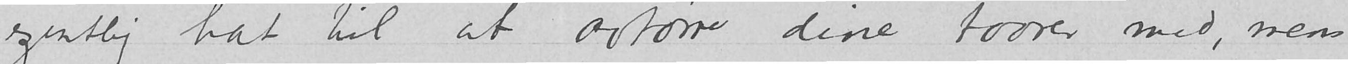egentlig hat til at avtørre dine taarer med, mens
